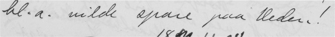bl.a. vilde spære paa Veden!
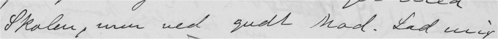Skolen, men ved godt Mod. Lad mig
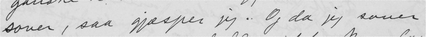sover, saa gjæsper jeg. Og da jeg sover
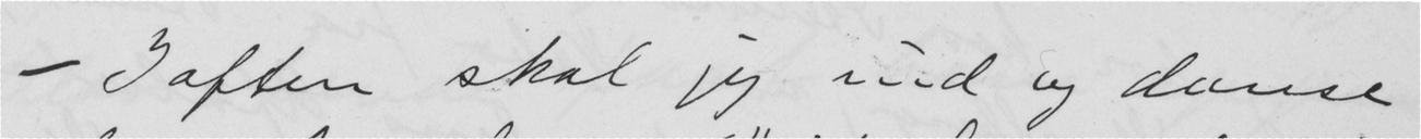— Iaften skal jeg ind og danse
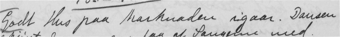Godt Hus paa Marknaden igaar. Dansen
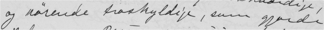og nørende troskyldige, som gjorde
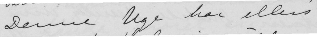Denne Uge har ellers
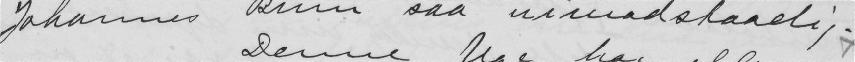Johannes Brun saa uimodstaaelig.
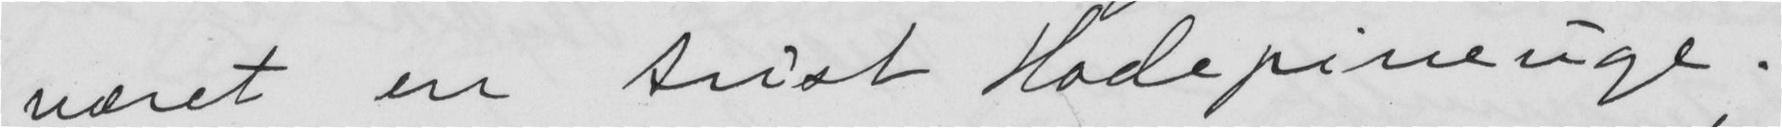været en trist Hodepineuge.
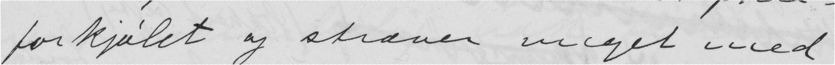forkjølet og stræver meget med
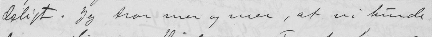deligt. Jeg tror mer og mer, at vi kunde
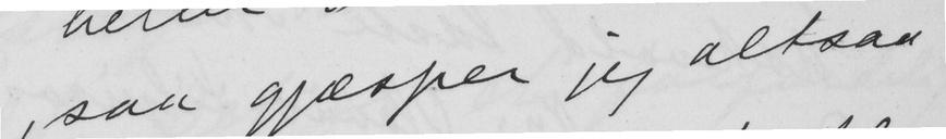, saa gjæsper jeg altsaa
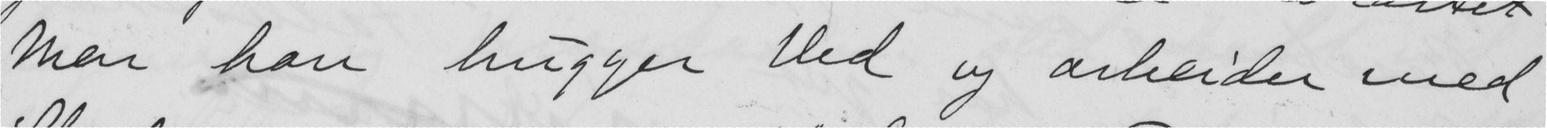Men han hugger Ved og arbeider med
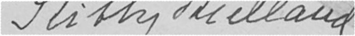Kitty Kielland
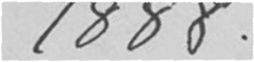1888.
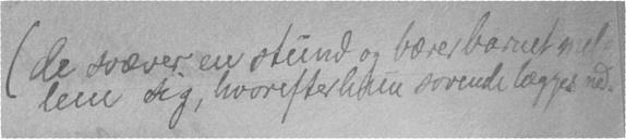(de svæver en stund og bærer barnet mel-lem sig, hvorefter hun sovende lægges ned.)
